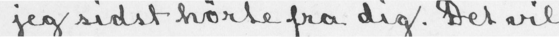jeg sidst hørte fra dig. Det vil
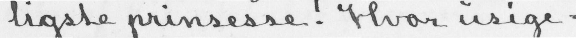ligste prinsesse! Hvor usige-
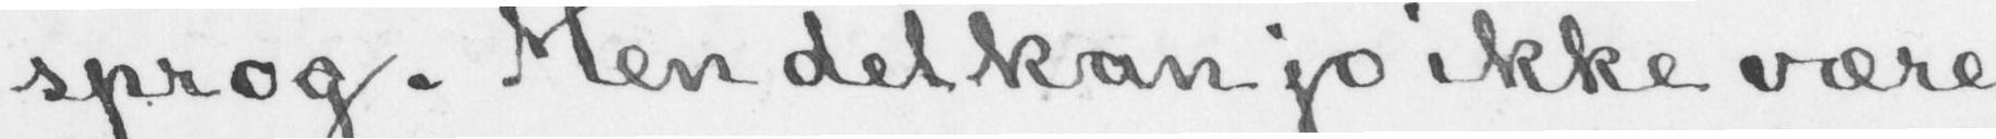sprog. Men det kan jo ikke være
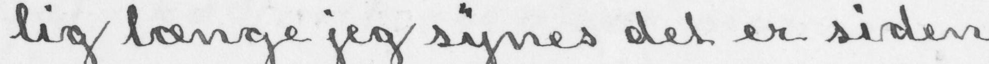lig længe jeg synes det er siden
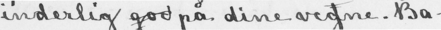inderlig god på dine vegne. Ba-
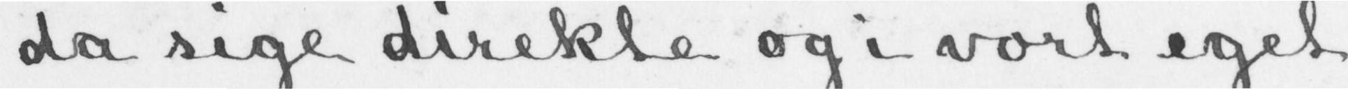da sige direkte og i vort eget
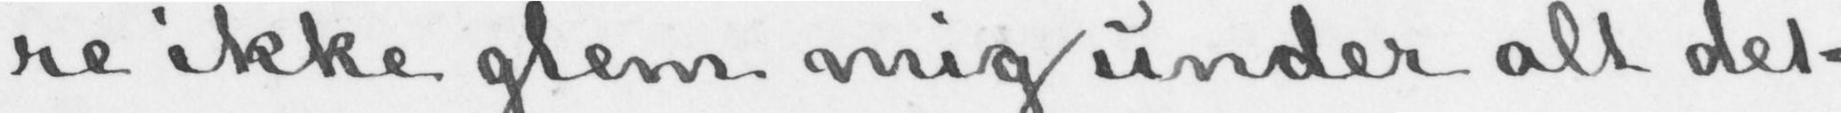re ikke glem mig under alt det-
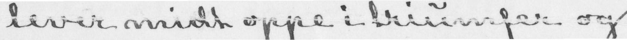lever midt oppe i triumfer og
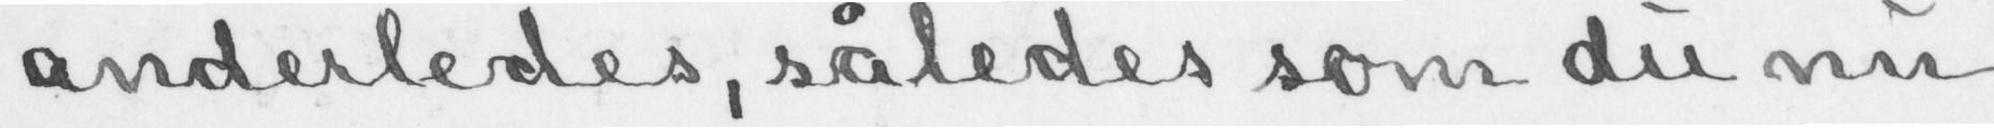anderledes, således som du nu
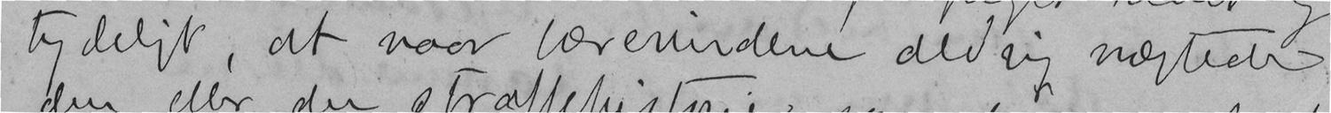tydeligt, at naar lærerindene aldrig nægtede
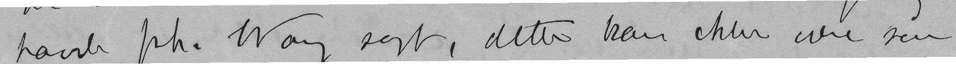havde frk. Wang sagt, dette kan ikke være san
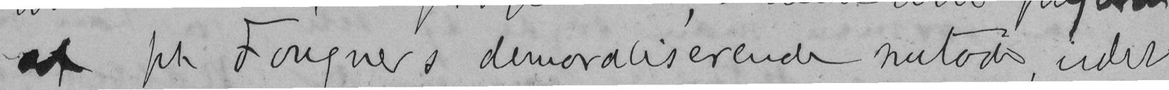af frk Fougners demoraliserende metode, idet
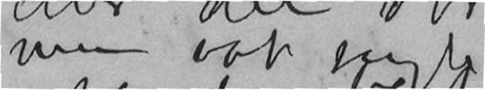men blot sagde
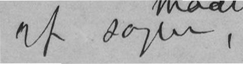af sagen,
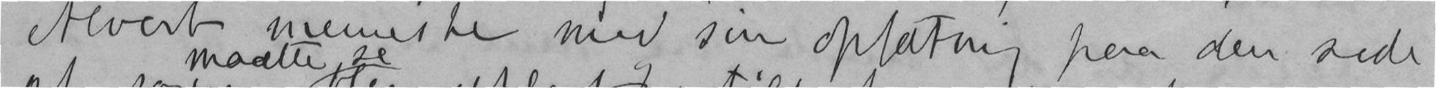ethvert menneske med sin opfatning paa den side
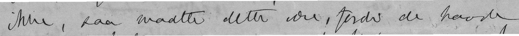ikke, saa maatte dette være, fordi de havde
